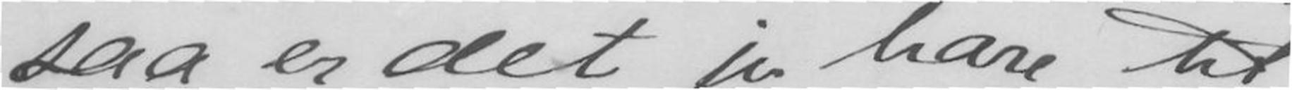saa er det jo bare til
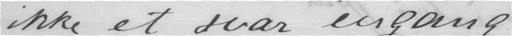ikke et svar engang.
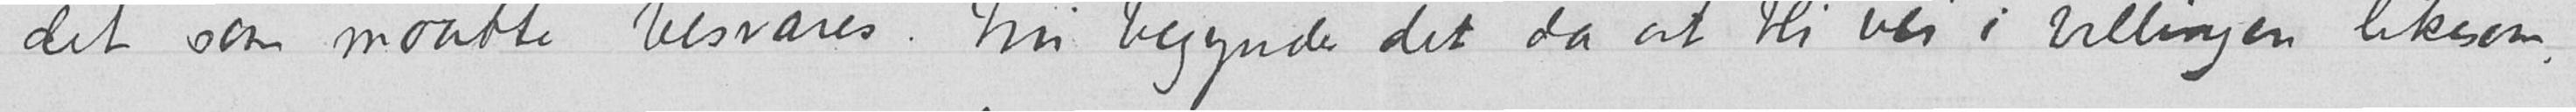det som maatte besvares. Nu begynder det da at bli vei i vellingen likesom,
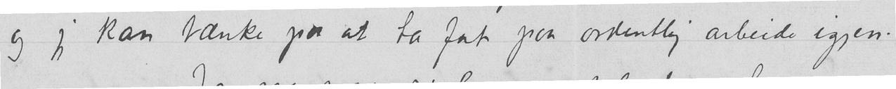og jeg kan tænke paa at ta fat paa ordentlig arbeide igjen.
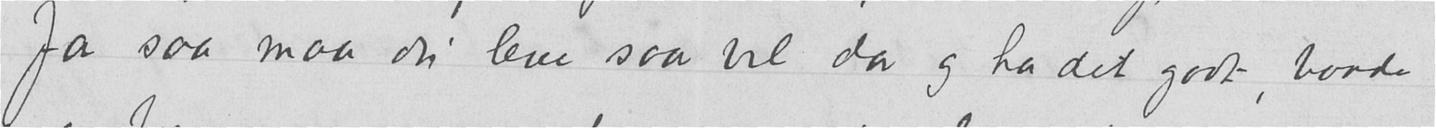Ja saa maa du leve saa vel da og ha det godt, baade
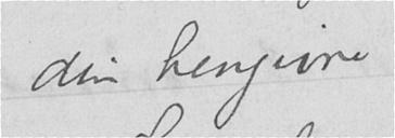din hengivne
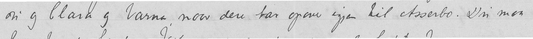du og Clara og barna, naar dere tar opover igjen til Asserbo. Du maa
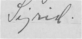Sigrid.
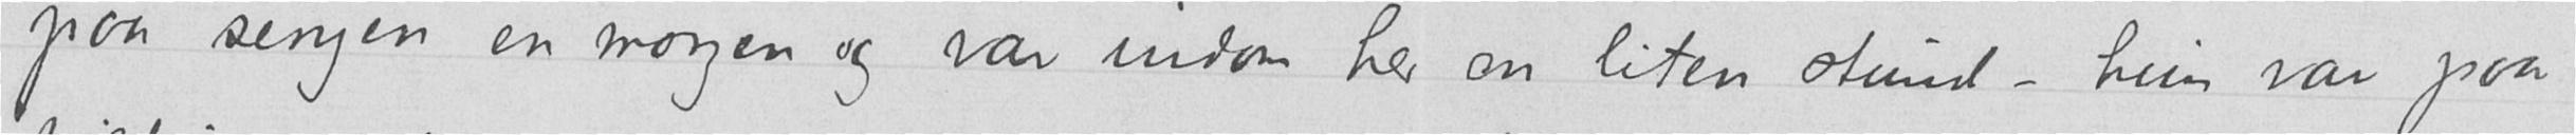paa sengen en morgen og var indom her en liten stund – hun var paa
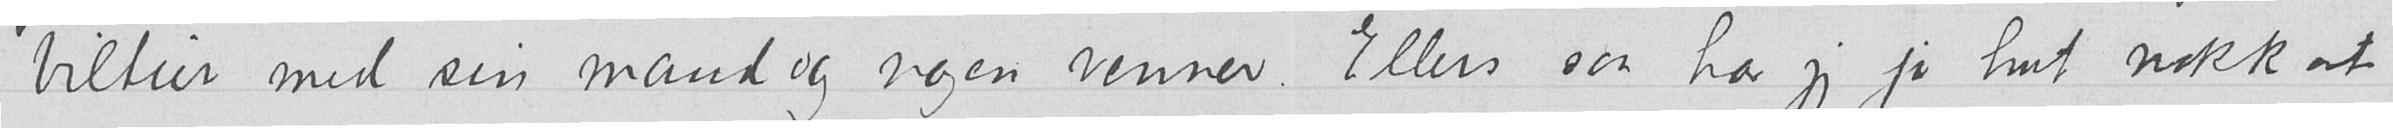biltur med sin mand og nogen venner. Ellers saa har jeg jo hat nokk at
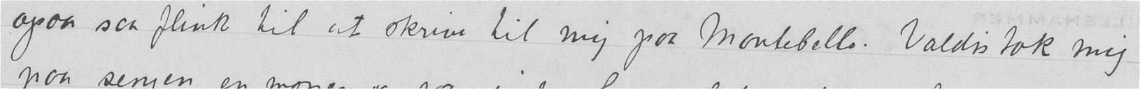ogsaa saa flink til at skrive til mig paa Montebello. Valdis tok mig
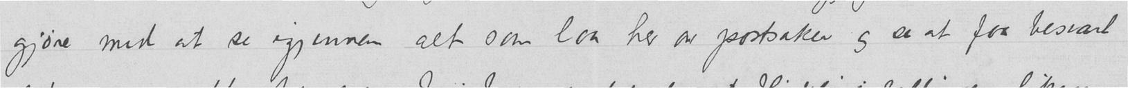gjøre med at se igjennem alt som laa her av postsaker og se at faa besvart
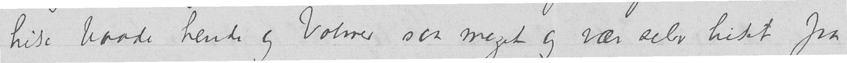hilse baade hende og Volmer saa meget og vær selv hilset fra
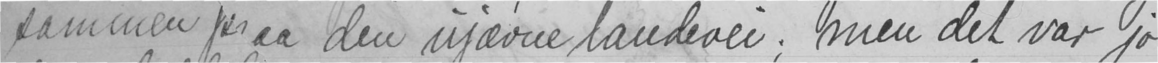sammen paa den ujævne landevei; men det var jo
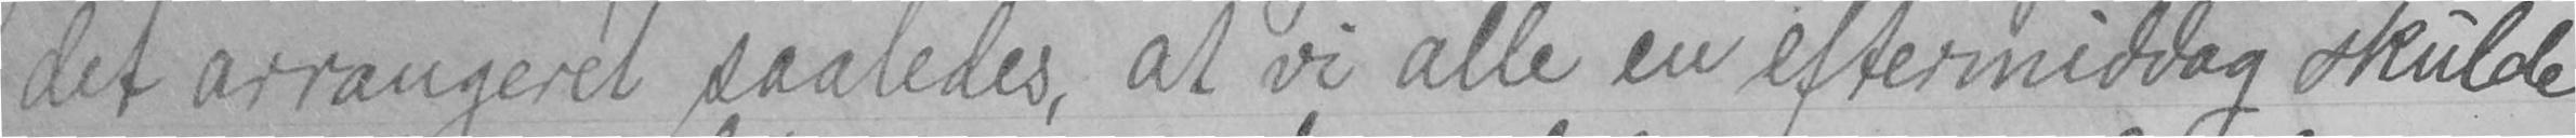det arrangeret saaledes, at vi alle en eftermiddag skulde
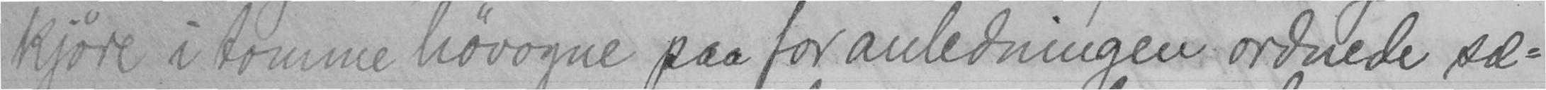kjøre i tomme høvogne paa for anledningen ordnede sæ-
